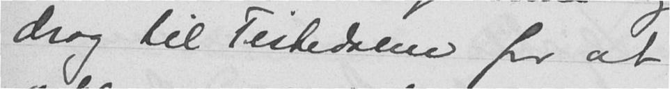drog til Tistedalen for at
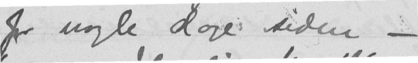for nogle dage siden -
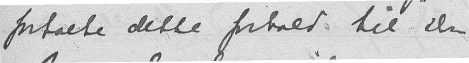fortalte dette forhold til Don
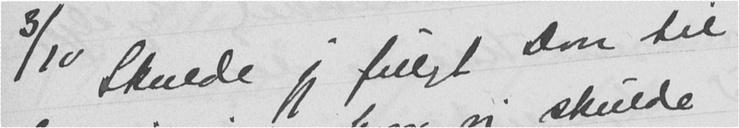3/10 Skulde jeg fulgt Don til
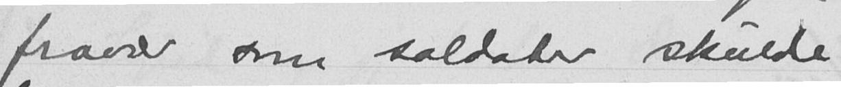fravær som soldater skulde
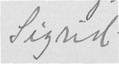Sigrid.
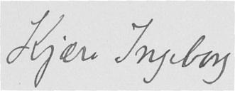Kjære Ingeborg
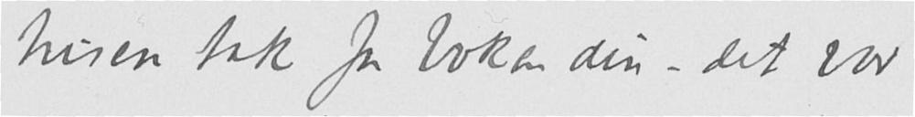tusen tak for boken din – det var
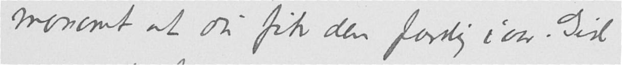morsomt at du fik den færdig iaar. Gid
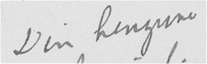Din hengivne
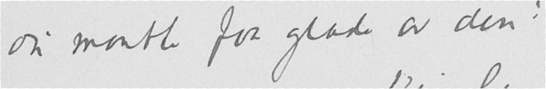du maatte faa glæde av den!
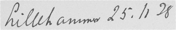Lillehammer 25.11 28
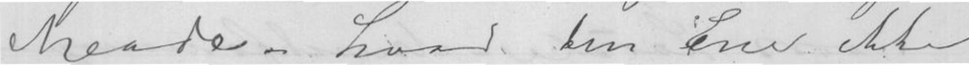Maade. hvad den Ene ikke
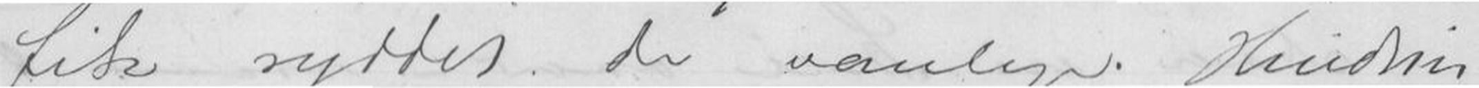fik ryddet de vanlige Hindrin
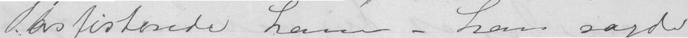assisterede ham - han sagde
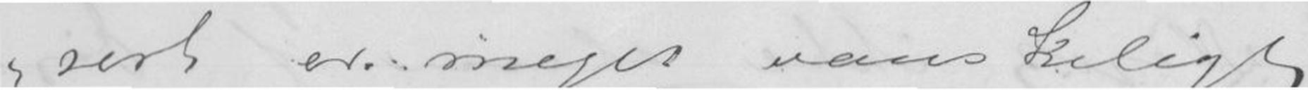sert er meget vanskelig
